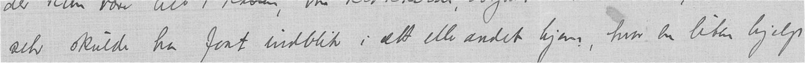selv skulde ha faat indblik i ætt eller andet hjem, hvor en liten hjelp
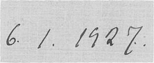6.1.1927
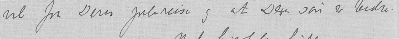vel fra Deres julereise og at Deres søn er bedre.
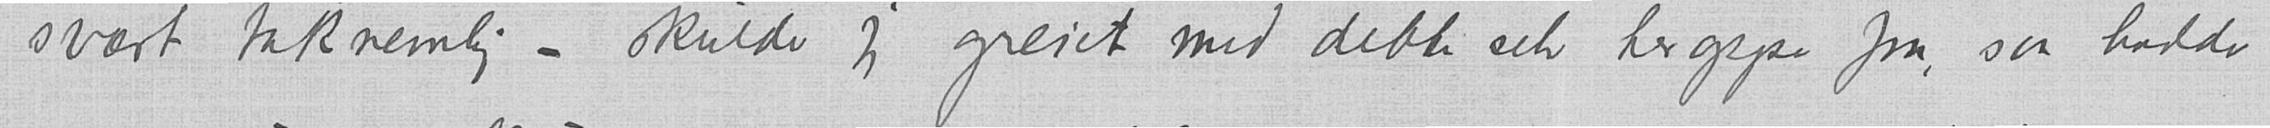svært taknemlig – skulde jeg greiet med dette selv her oppe fra, saa hadde
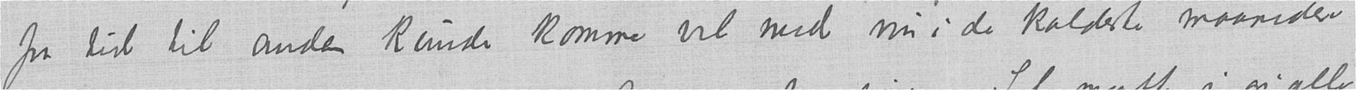fra tid til anden kunde komme vel med nu i de kaldeste maaneder
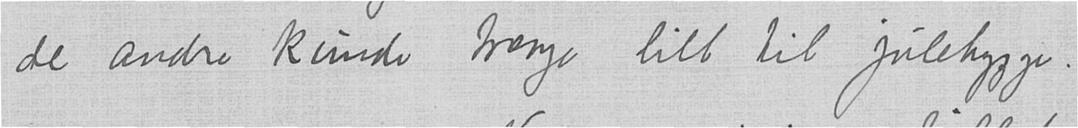de andre kunde trænge litt til julehygge.
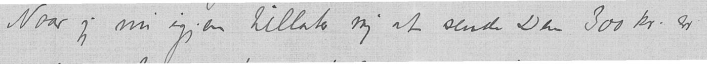Naar jeg nu igjen tillater mig at sende Den 300 kr. er
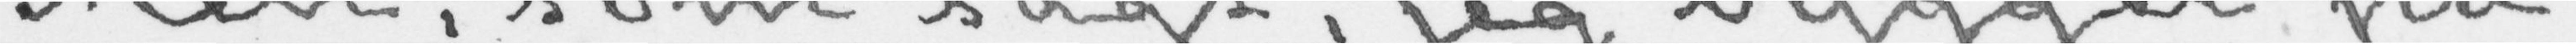Men, som sagt, jeg bygger på
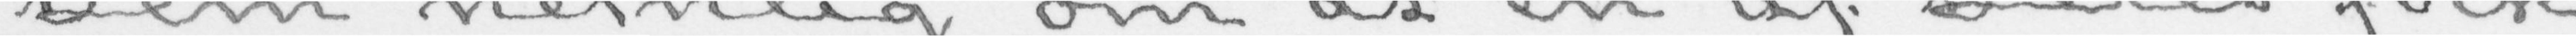Dem nemlig om at en af Deres folk
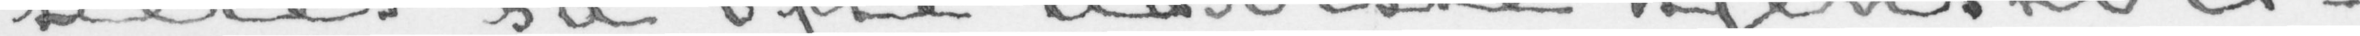Deres så ofte udviste tjenstvil-
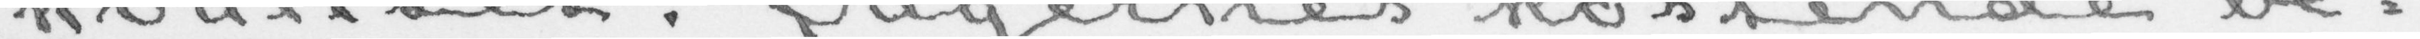kvalitet. Sagernes kostende be-
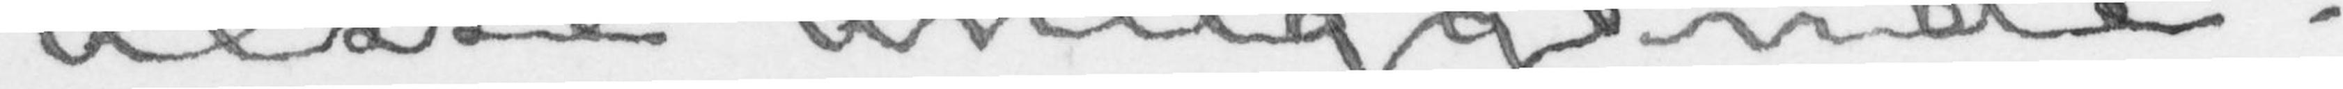dette anliggende.
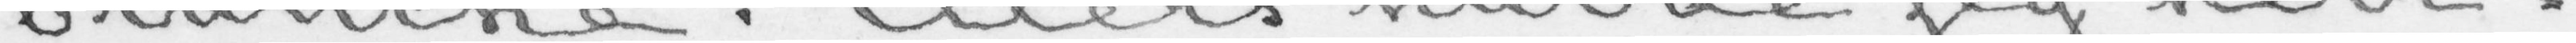branche. Ellers havde jeg hen-
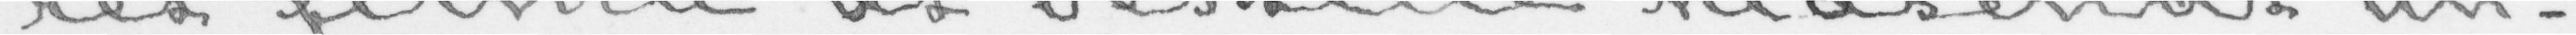ret firma at bestille hidsendt un-
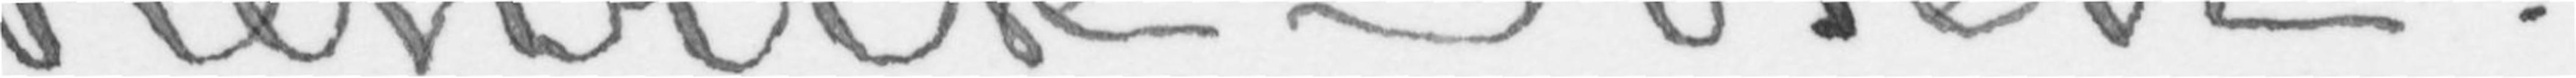Henrik Ibsen.
